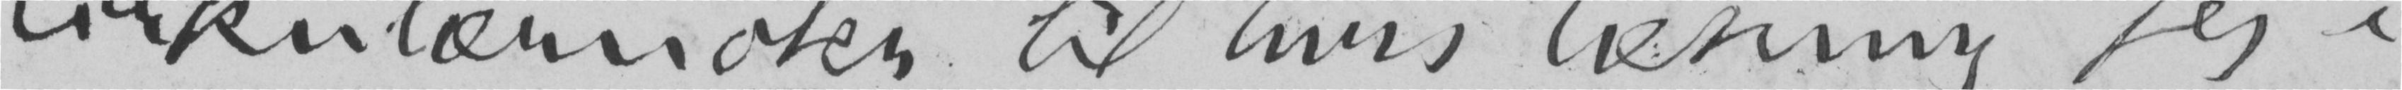cirkulærnoter, til hvis læsning jeg i
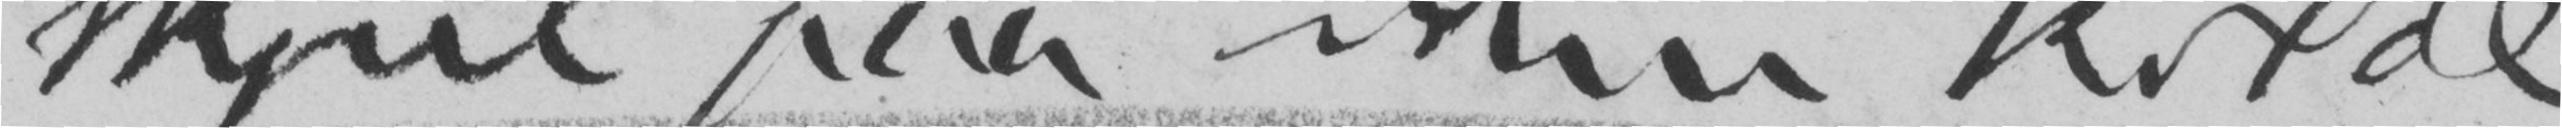skjul paa min kilde.
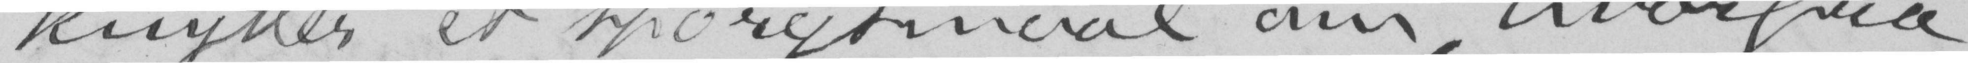knytter et spørgsmaaal om, hvorfra
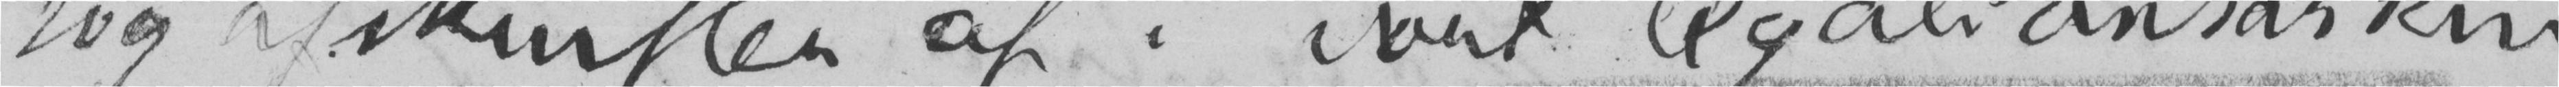tog afskrifter af i vort legationsarkiv
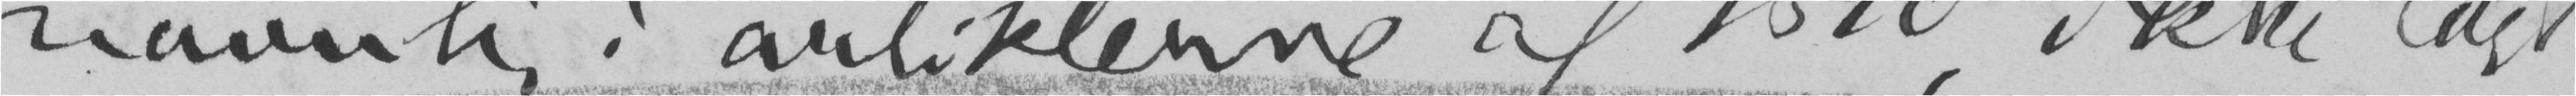navnlig i artiklerne af 1890, ikke lagt
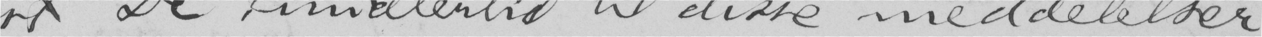De imidlertid til disse medddelser
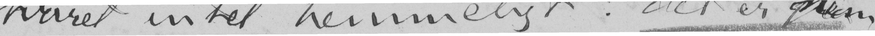svaret intet hemmeligt: det er nem-
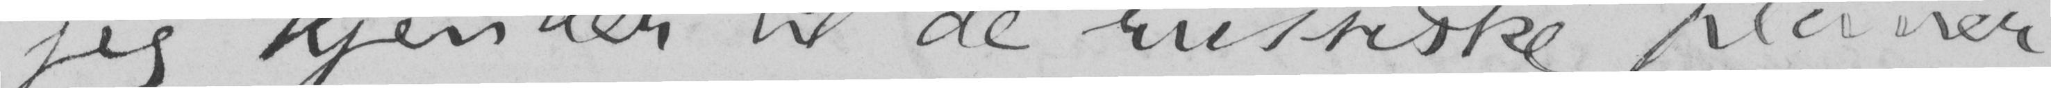jeg kjender til de russiske planer
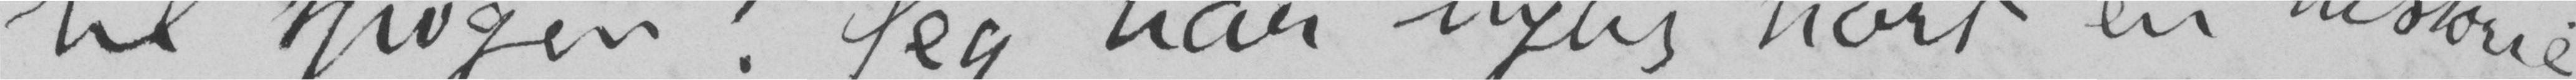til spøgen! Jeg har nylig hørt en historie
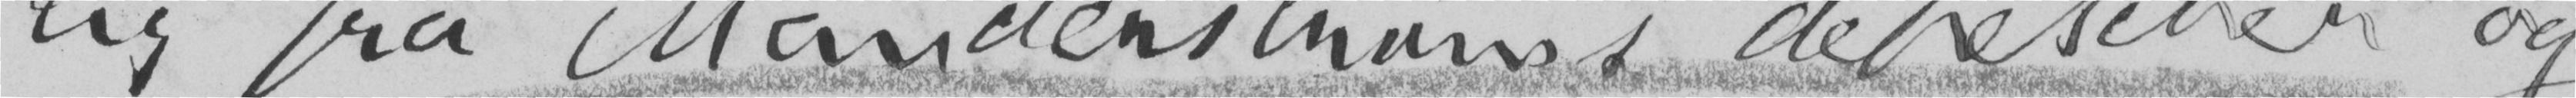lig fra Mandenströms depescher og
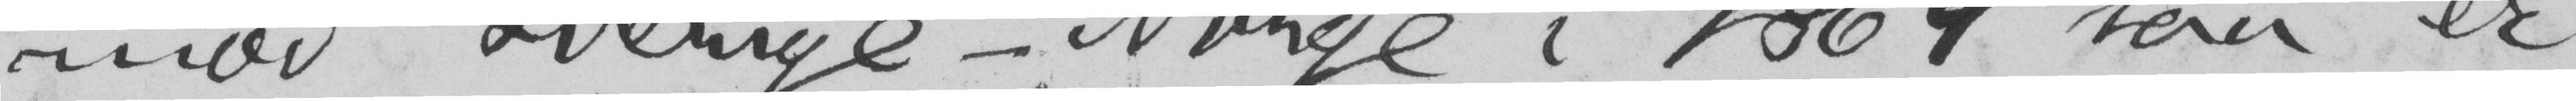mod Sverige-Norge i 1864, saa er
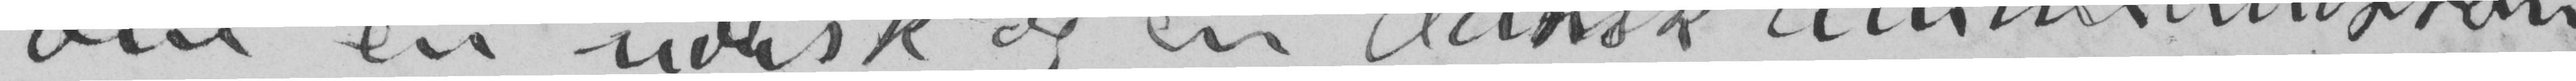om en norsk og en dansk amtmandssøn,
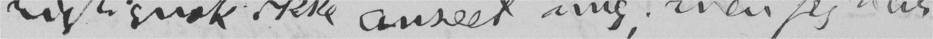rigtignok ikke anseet mig; men jeg har,
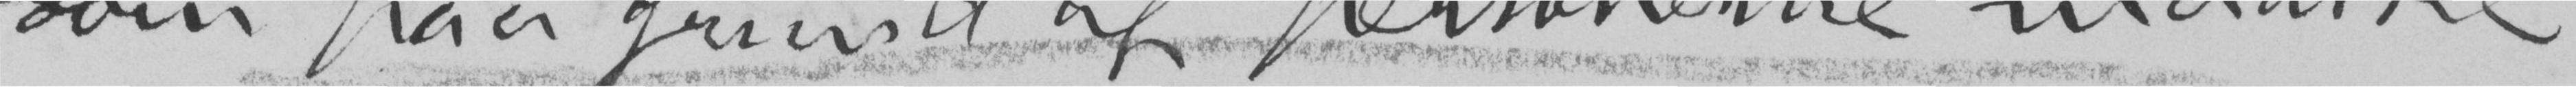som paa grund af personerne maaaske
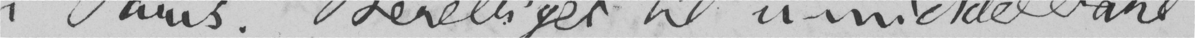i Paris. Berettiget til umiddelbart
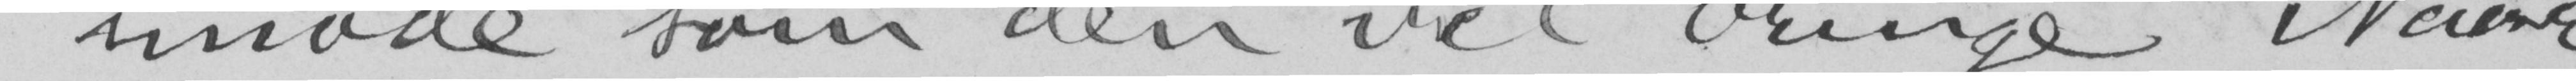imøde, som den vil bringe. Naar
